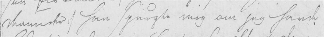derunder :/ han spurgte mig om jeg havde
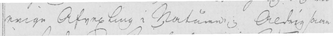evige Afvexling i Naturen; Aldrig saae
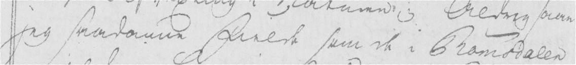jeg saadanne Fielde som de i Romsdalen
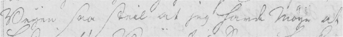Veyen saa steil at jeg havde Møye at
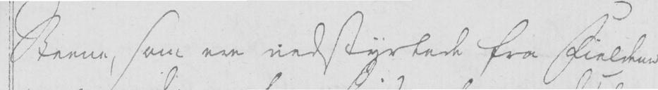Steene, som ere nedstyrtede fra Fieldene
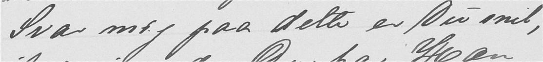Svar mig paa dette er Du snil,
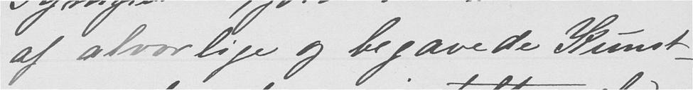af alvorlige og begavede Kunst-
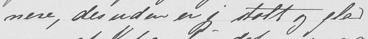nere, des uden er jeg stolt og glad
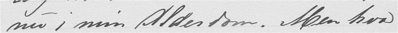nu i min Alderdom. Men hvad
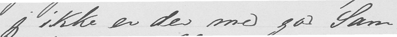jeg ikke er der med god Sam-
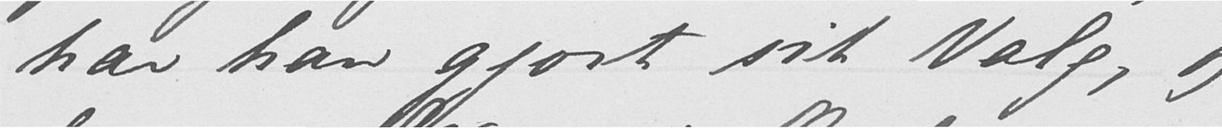har han gjort sit Valg, og
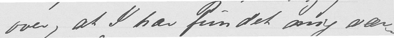over, at I har fundet mig vær-
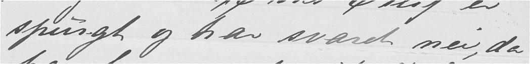spurgt og har svaret nei, da
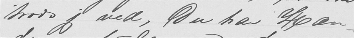trods jeg ved, Du har Hæn-
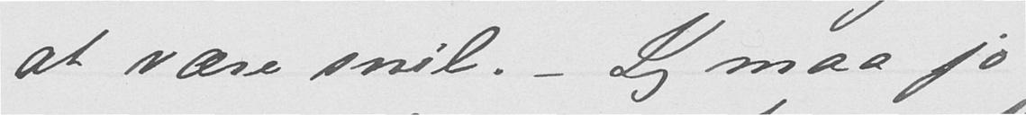at være snil. - Jeg maa jo
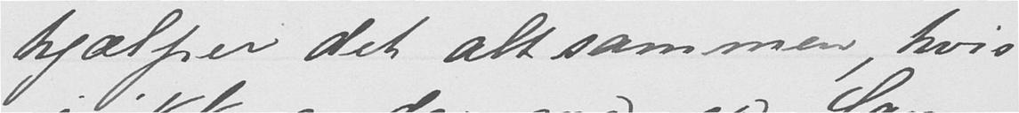hjælper det alt sammen, hvis
